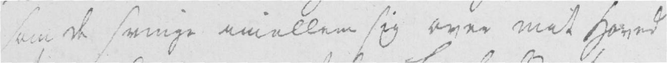som de svinge imellem sig over mit Hoved
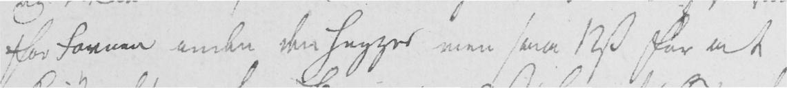for favnen andre den hugges men saa 12 s for at
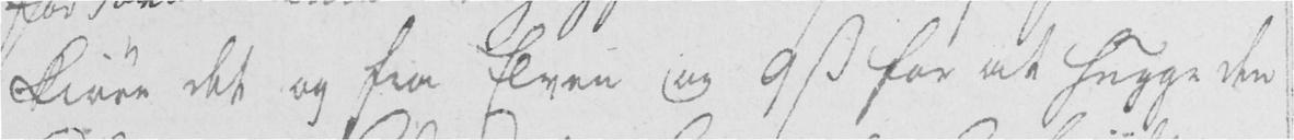kiøre det og fra Elven og 9 s for at hugge den.
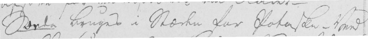Særde bruges i Stæde for Potaske-Veed
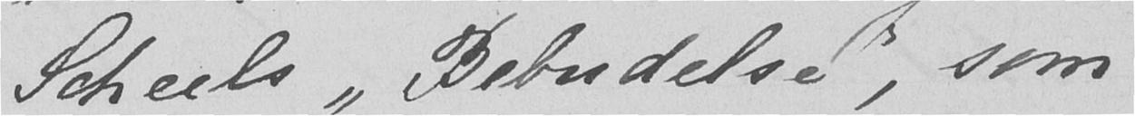Scheels "Bebudelse", som
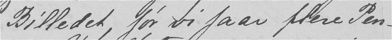Billedet, før vi faar flere Pen-
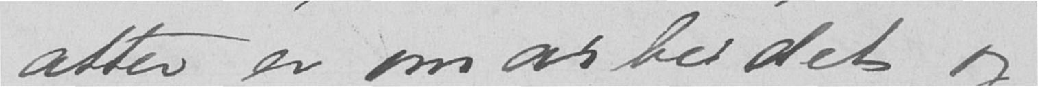atter er omarbeidet og
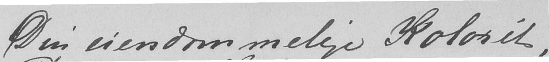Din eiendommelige Kolorit,
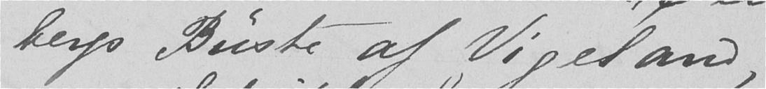bergs Buste af Vigeland,
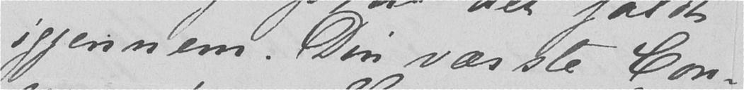igjennem. Din værste Con-
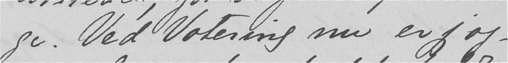ge. Ved Votering nu er jeg og-
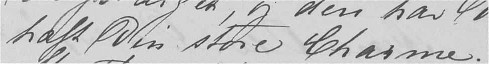haft Din store Charme.
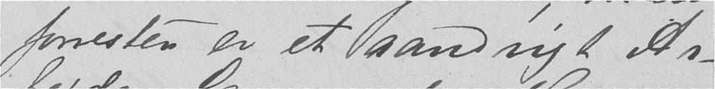forresten er et aandrigt Ar-
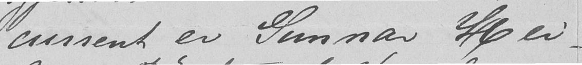current er Gunnar Hei-
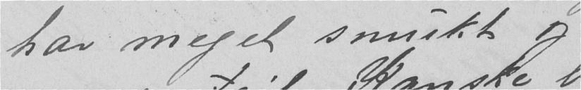har meget smukt og
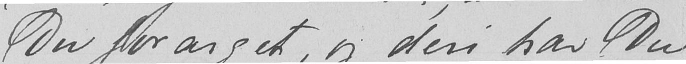Du forarget, og deri har Du
